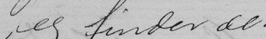Jeg finder de
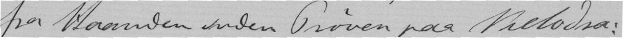fra Haanden inden Praven paa Melodra:
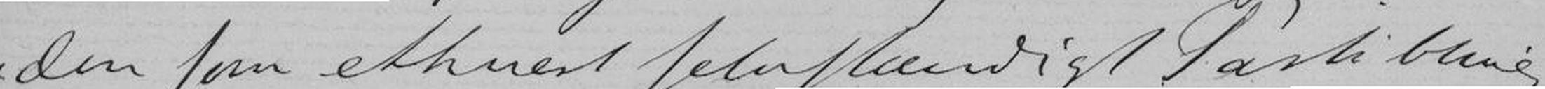=den som ethvert selvstændigt Parti bliver
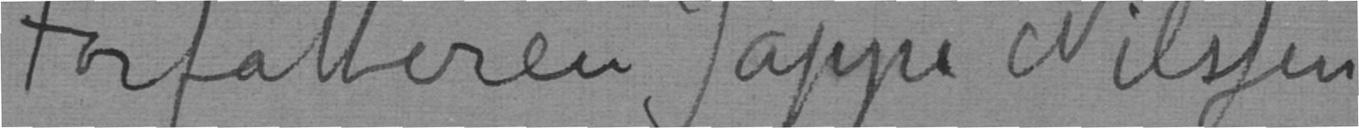Forfatteren Jappe Nilssen
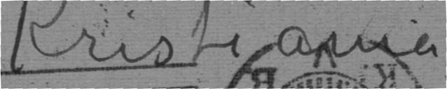Kristiania
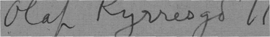Olaf Kyrresgd 11
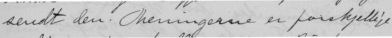sendt den. Meningerne er forskjellige
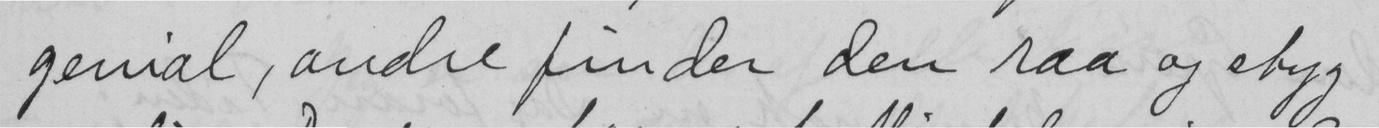genial, andre finder den raa og styg
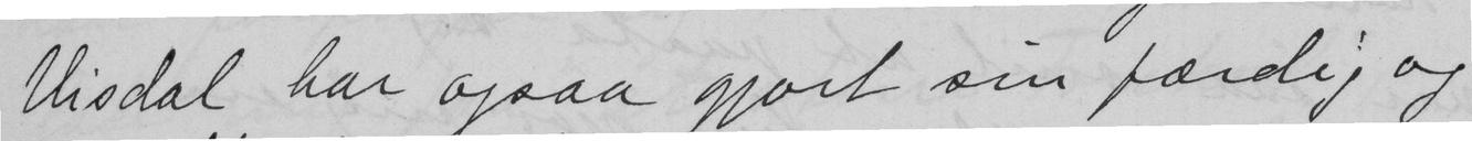Visdal har ogsaa gjort sin færdig og
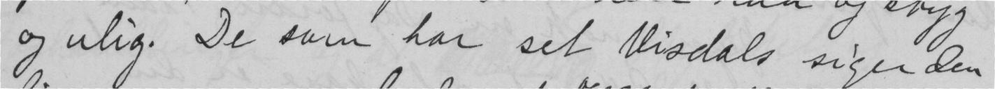og ulig. De som har set Visdals siger den
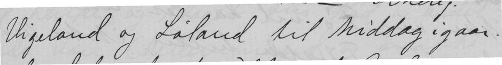Vigeland og Løland til Middag igaar.
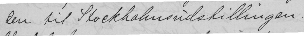den til Stockholmsudstillingen.
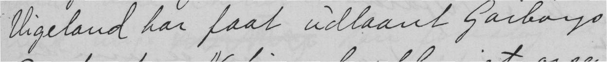Vigeland har faat udlaant Garborgs
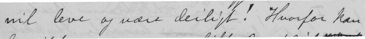vil leve og være deiligt! Hvorfor kan
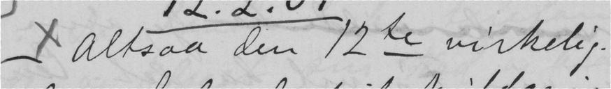x Altsaa den 12te virkelig.
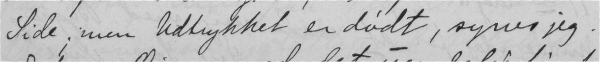Side; men Udtrykket er dødt, synes jeg.
